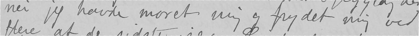nei jeg havde moret mig og frydet mig ved
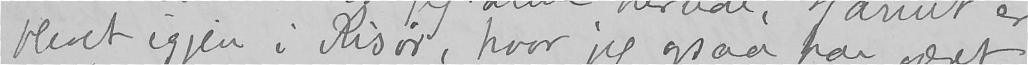blevet igjen i Risør, hvor jeg ogsaa har været
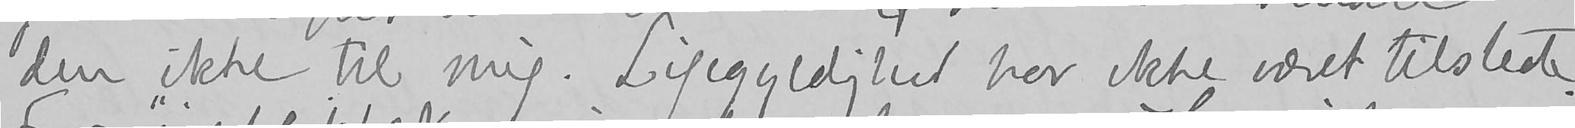den ikke til mig. Ligegyldighed har ikke været tilstede.
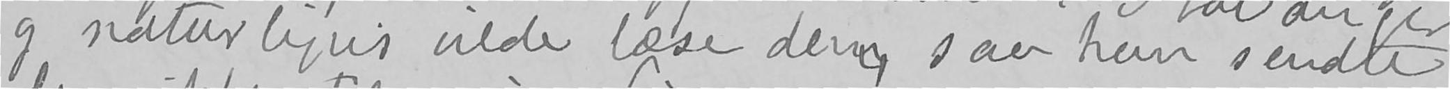og naturligvis vilde læse den, saa hun sendte
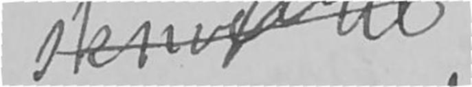skrive til
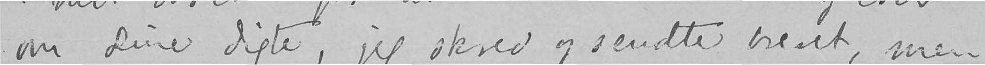om dine digte, jeg skrev og sendte brevet, men
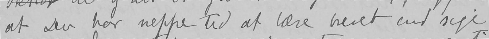at du har neppe tid at læse brevet end sige
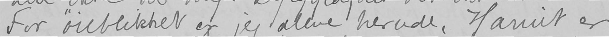For øieblikket er jeg alene herude, Harriet er
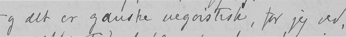og det er ganske uegoistisk, for jeg ved,
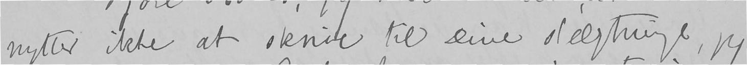nytter ikke at skrive til dine slægtninge, jeg
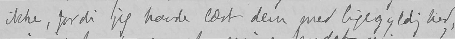ikke, fordi jeg havde læst dem med ligegyldighed,
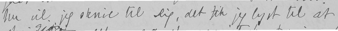Nu vil jeg skrive til dig, det fik jeg lyst til at
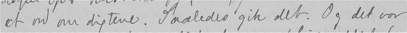et ord om digtene. Saaledes gik det. Og det var
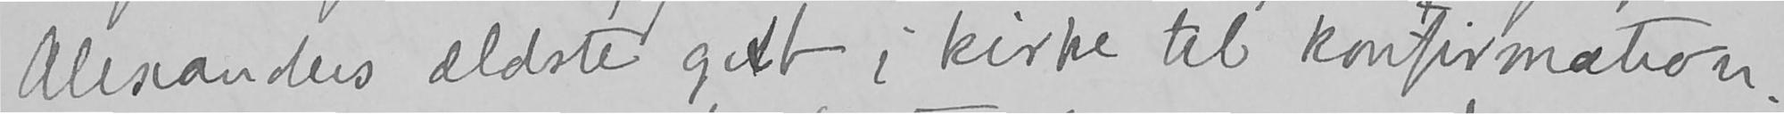Alexanders ældste gut i kirke til konfirmation.
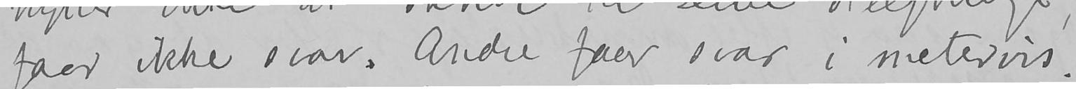faar ikke svar. Andre faar svar i metervis.
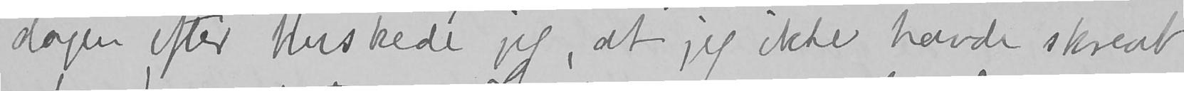dagen efter huskede jeg, at jeg ikke havde skrevet
